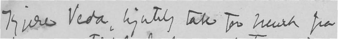Kjære Veda, hjertelig tak for brevet fra
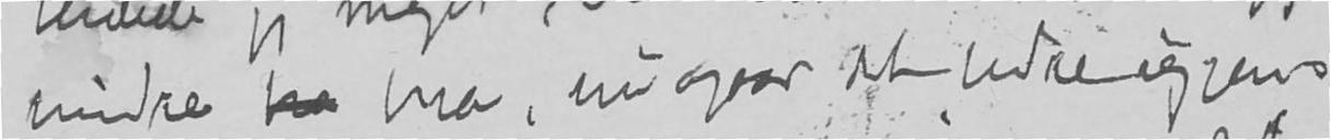mindre ha bra, nu ogsaa det bedre igjen.
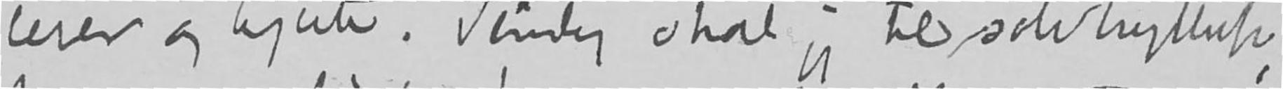lever og hjerte. Sinding skal jeg til sølvbryllup,
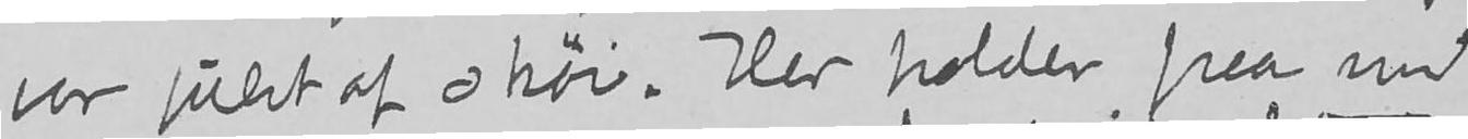var fuldt af skøi. Her holder paa med
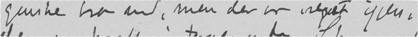ganske bra med, men der er meget igjen,
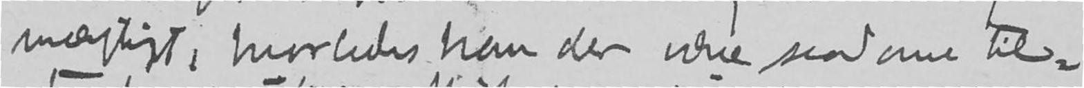mægtigt, hvorledes kan der være saadane til-
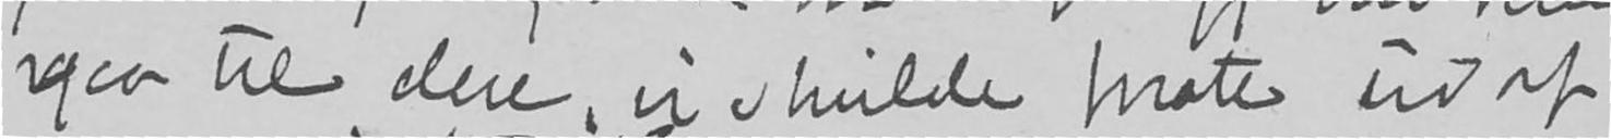gaa til dere, vi skulde prate ud af
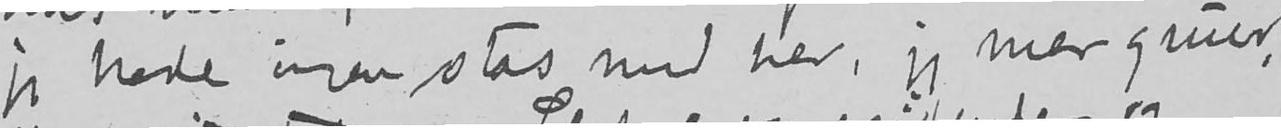jeg havde ingen stas med her, jeg mer gruer,
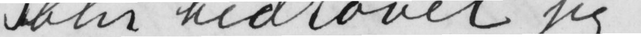blir bedrøvet jeg
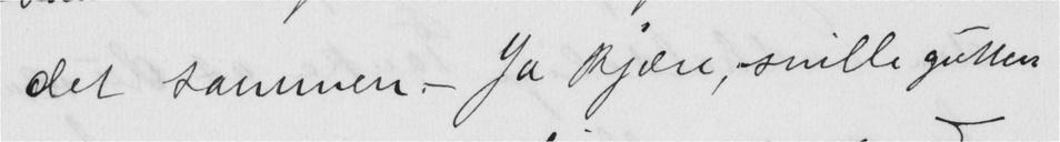det sammen- Ja Kjære, snille gutten
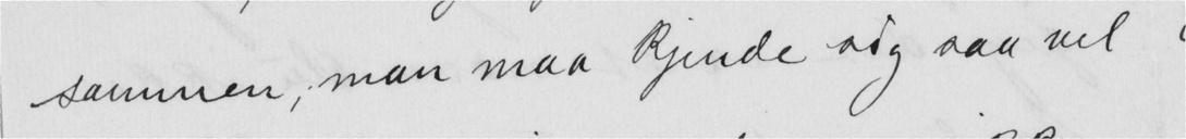sammen, man maa kjende sig saa vel
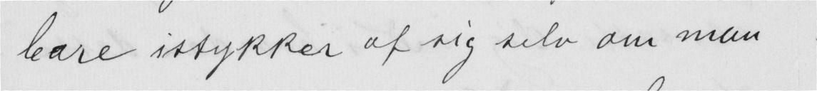bare istykker af sig selv om man
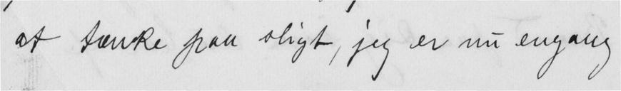at tænke paa sligt, jeg er nu engang
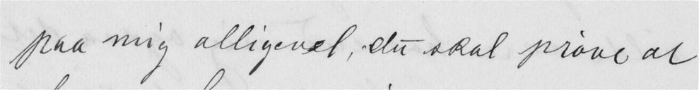paa mig alligevel, du skal prøve at
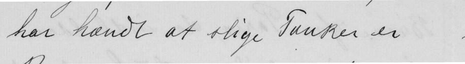har hendt at slige Tanker er
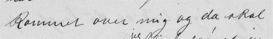Kommet over mig og da skal
